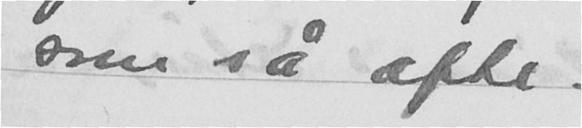som så ofte.
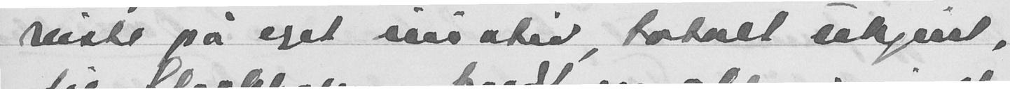reiste på eget iniativ, totalt ukjent,
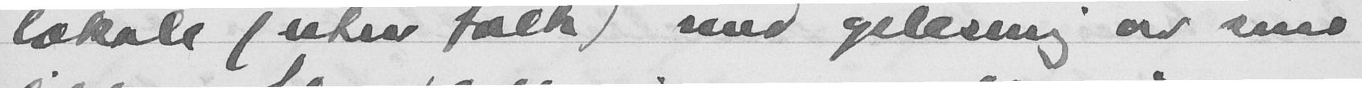lokale (uten folk) med oplesning av sine
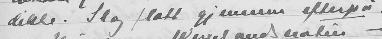dikte. Slog flatt gjennom efterpå.
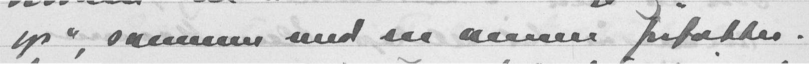op", sammen med en annen forfatter.
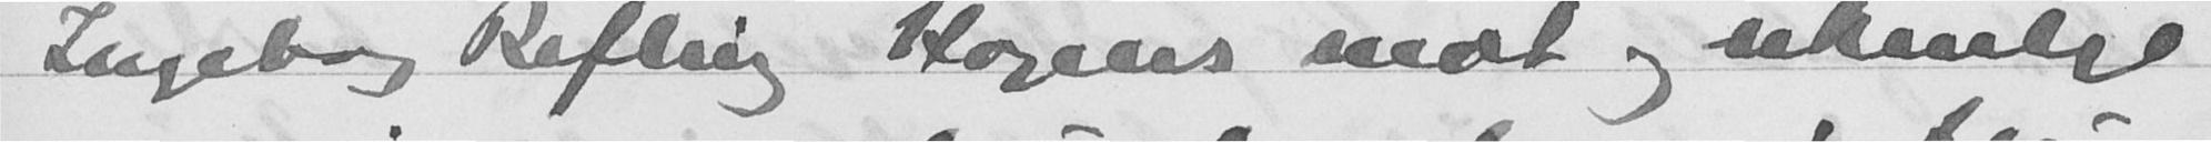Ingeborg Refling Hagens mot og ukuelige
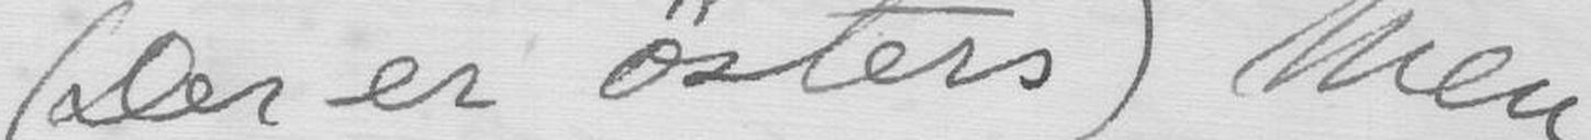(Der er østers) Men
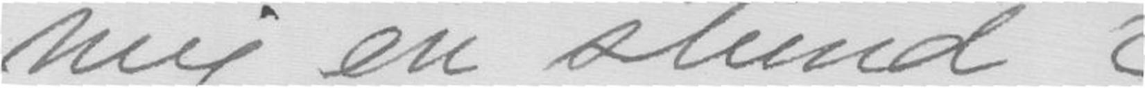mig en stund?
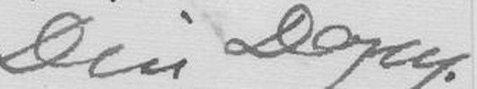Din Dagny.
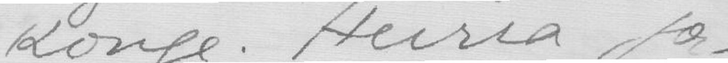Konge. Hurra for
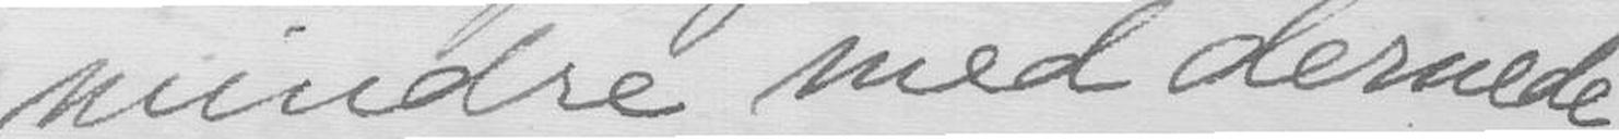mindre med dernede
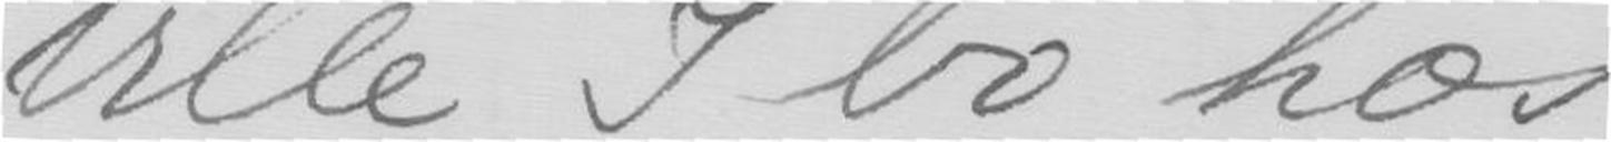Ville I bo hos
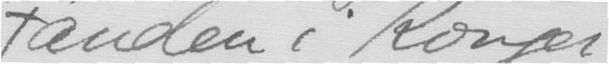Fanden i Konger!
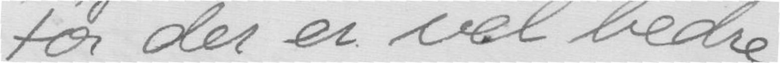For der er vel bedre
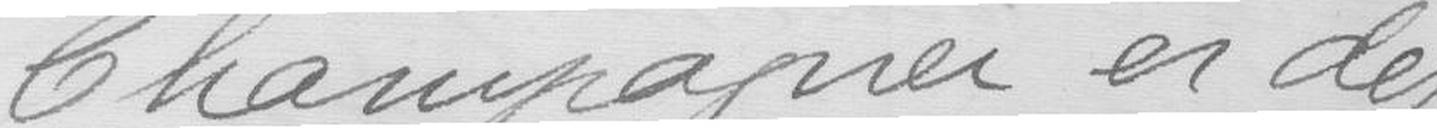Champage er det
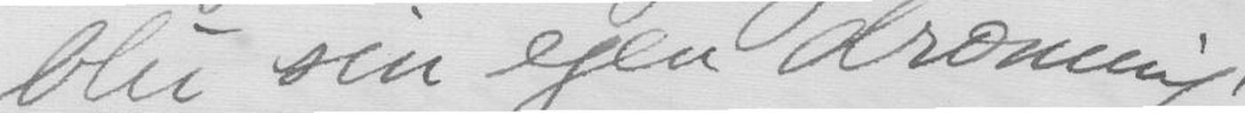blir sin egen dronning!
